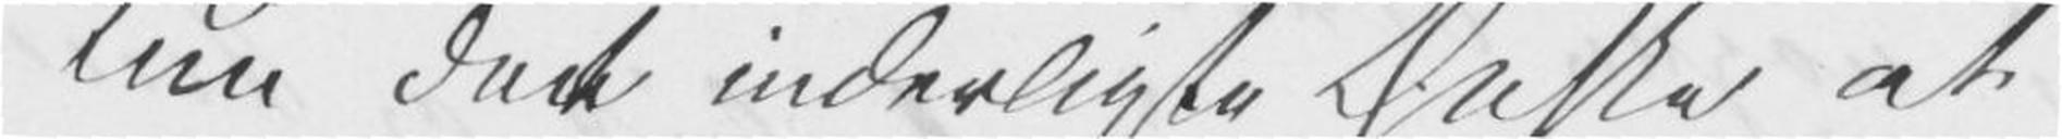Kun det inderligste Ønske at
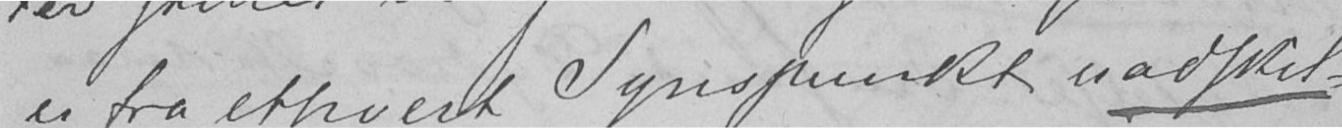er fra ethvert Synspunkt uadskil-
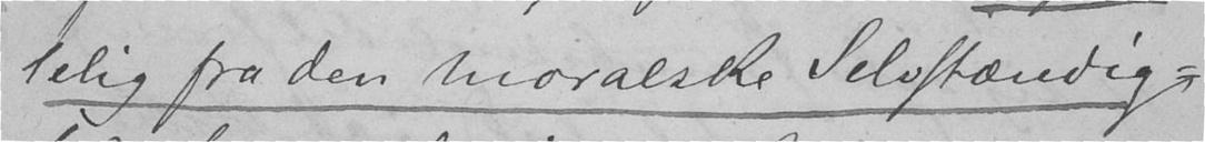lelig fra den moralske Selvstændig-
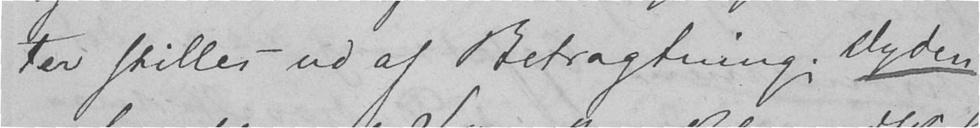ter stilles ud af Betragtning. Dyden
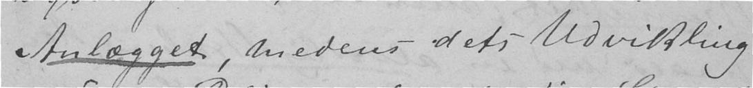Anlægget, medens dets Udvikling
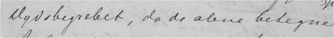Dydsbegrebet, da de alene betegne
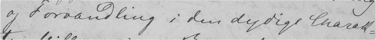og Forvandling i den dydige Charak-
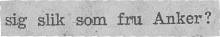sig slik som fru Anker?
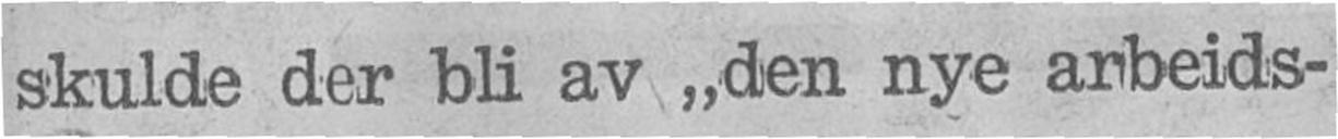skulde der bli av "den nye arbeids-
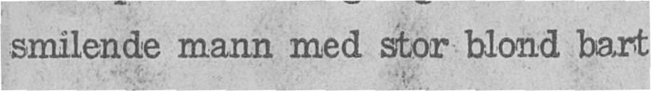smilende mann med stor blond bart
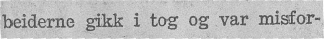beiderne gikk i tog og var misfor-
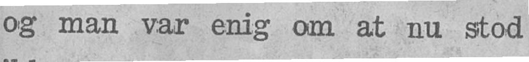og man var enig om at nu stod
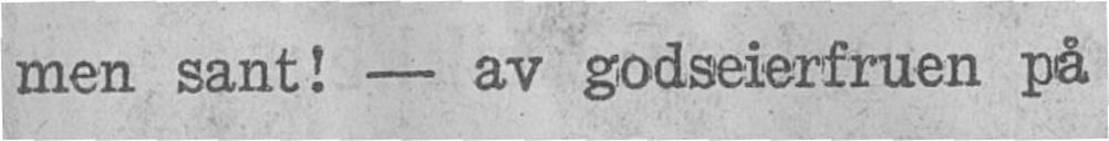men sant! - av godseierfruen på
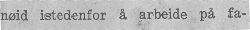nøid istedenfor å arbeide på fa-
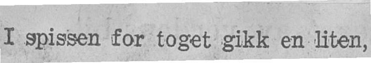I spissen for toget gikk en liten,
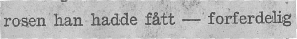rosen han hadde fått - forferdelig
